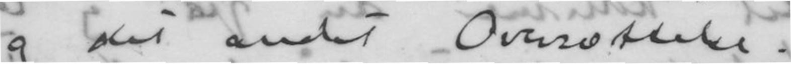og det Landet Oversættelse.
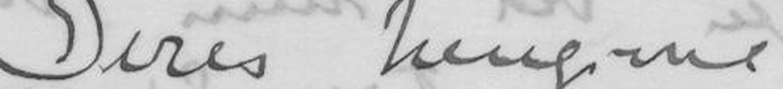Deres hengivne
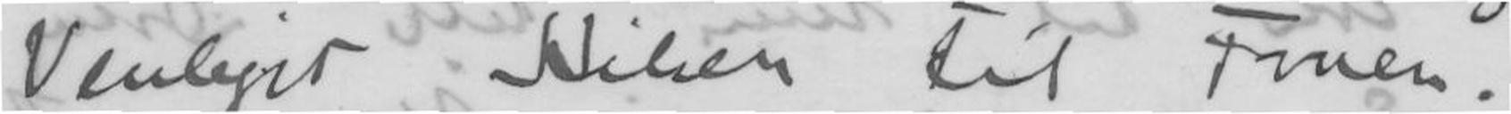Venligst Hilsen til Fruen.
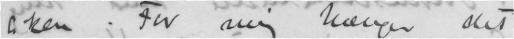siken. For mig hænger det
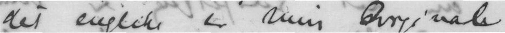det engelske er min Orginale
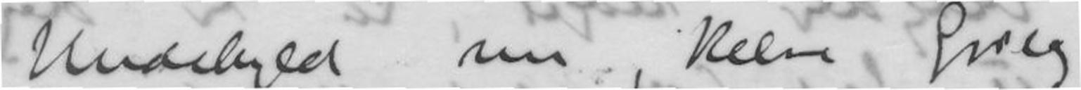Undskyld nu kære Grieg,
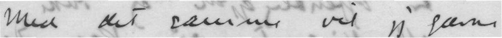Med det samme vil jeg gærne
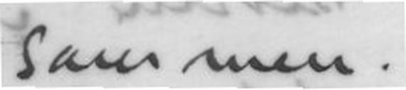sammen.
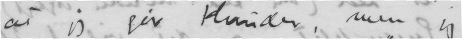at jeg gir Hunder, men jeg
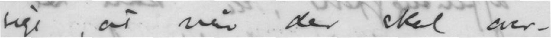sige, at når der skal over-
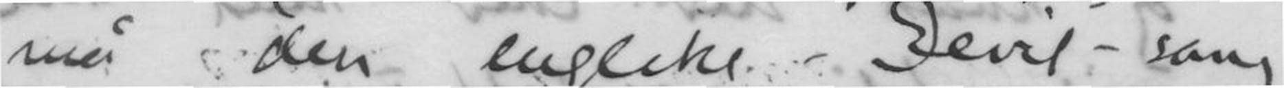må den engelske - Devil - sang
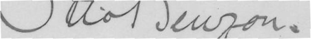Otto Benzon
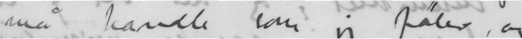må handle som jeg føler, og
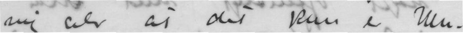mig selv at det kun er Mu-
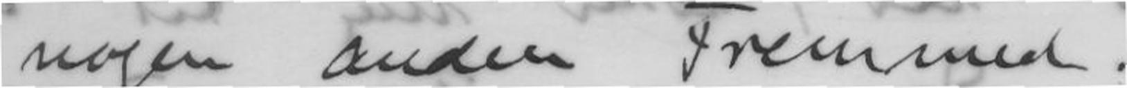nogen anden Fremmed.
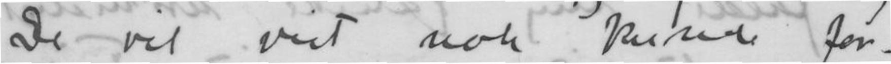De vil vist nok kunde for-
